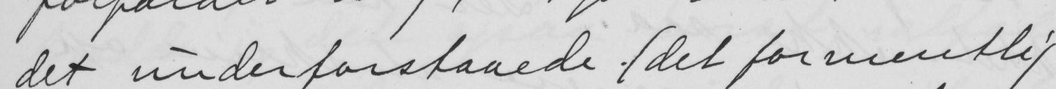det underforstaaede (det formentlig
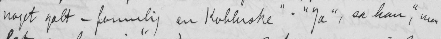noget galt — formulig en Koblerske". "Ja", sa han, "men
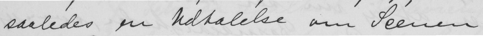saaledes en Udtalelse om Scenen
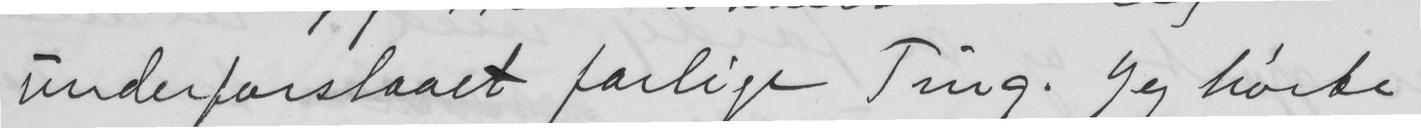underforstaaet farlige Ting. Jeg hørte
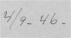4/9. 46.
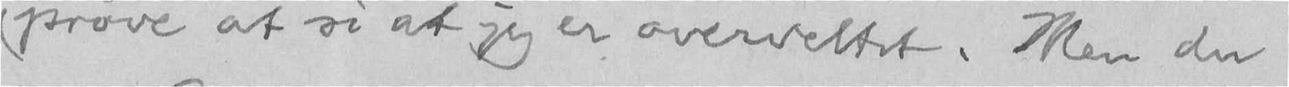prøve at si at jeg er overveltet. Men du
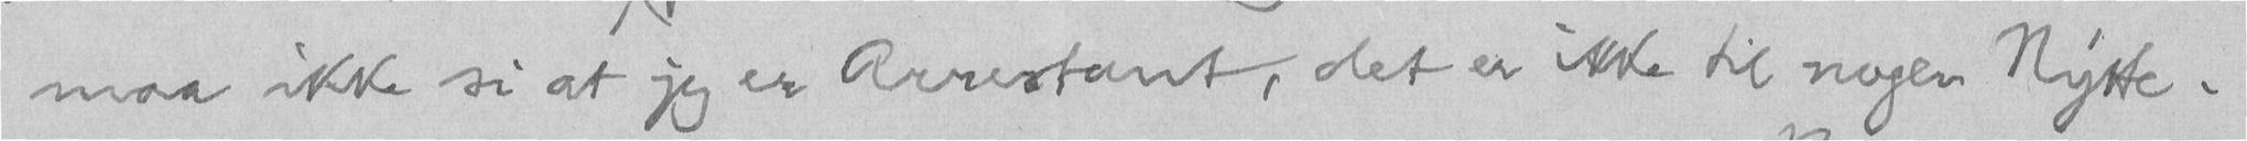maa ikke si at jeg er Arrestant, det er ikke til nogen Nytte.
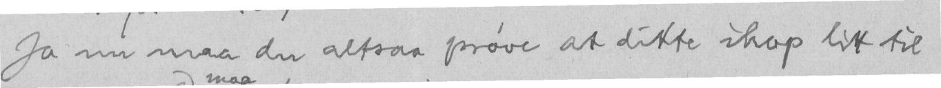Ja nu maa du altsaa prøve at dikte ihop litt til
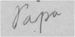Papa
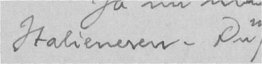Italieneren. Du
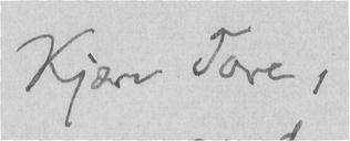Kjære Tore,
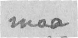maa
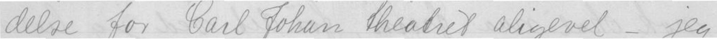delse for Carl Johan theatret aligevel - jeg
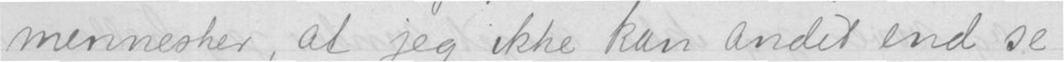mennesker, at jeg ikke kan andet end se
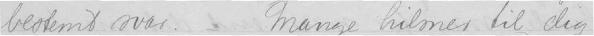bestemt svar. - Mange hilsner til dig
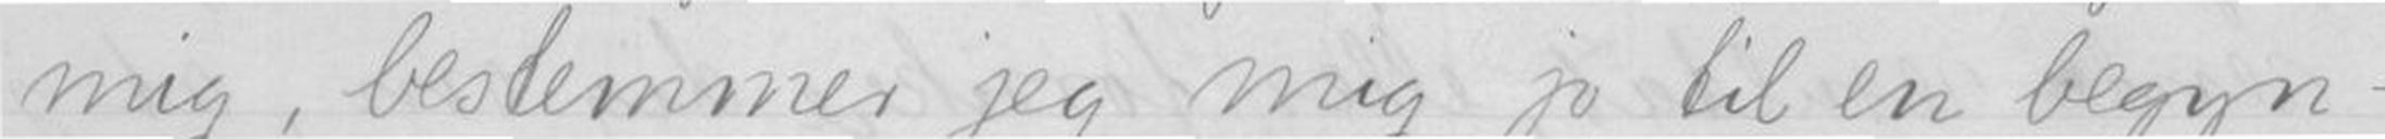mig, bestemmer jeg mig jo til en begyn-
